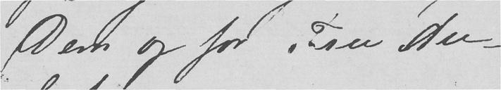Dem og for Fru Au-
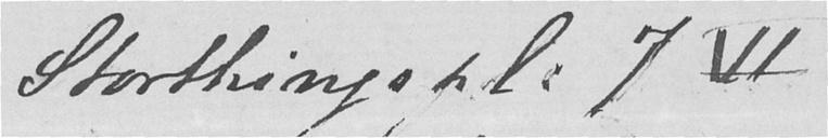Storthingspl. 7 VI
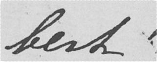bert!
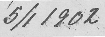5/1 1902
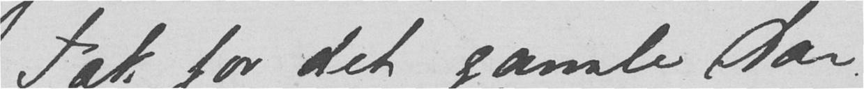Tak for det gamle Aar
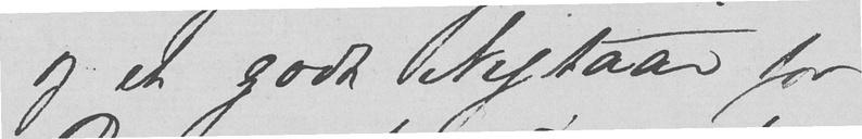og et godt Nytaar for
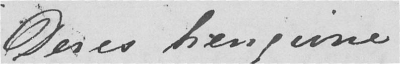Deres hengivne
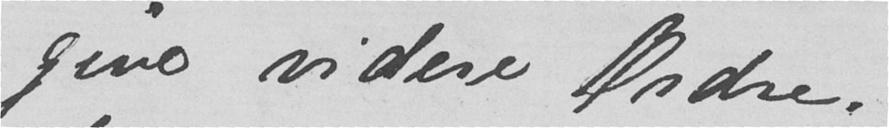give videre Ordre.
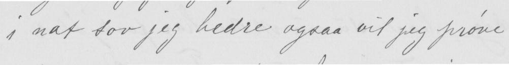i nat sov jeg bedre ogsaa vil jeg prøve
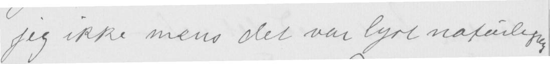jeg ikke mens det var lyst naturligvis
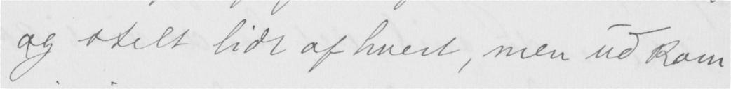og stelt lidt af hvert, men ud kom
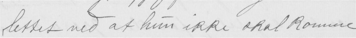lettet ved at hun ikke skal komme
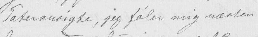Tateransigte, jeg føler mig næsten
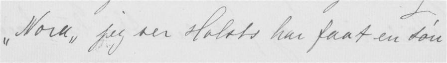"Nora" jeg ser Holsts har faat en søn
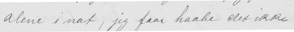alene i nat, jeg faar haabe det ikke
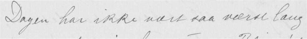Dagen har ikke vært saa værst lang
